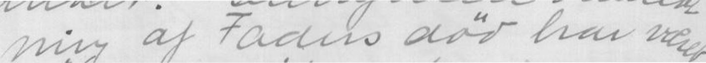ning af Faders død har været
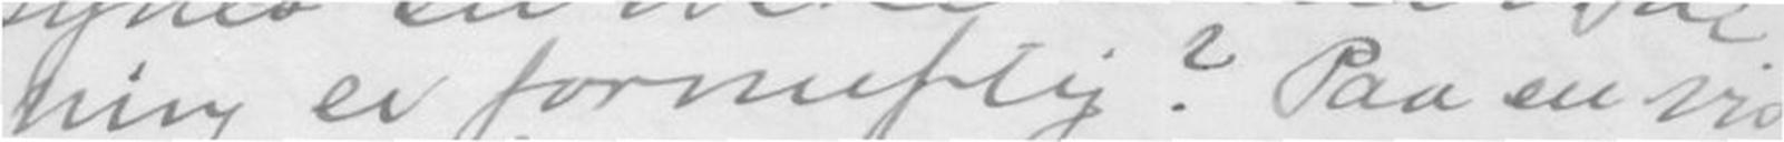ning er fornuftig? Paa en vis
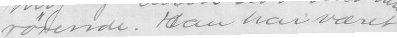rørende. Han har været
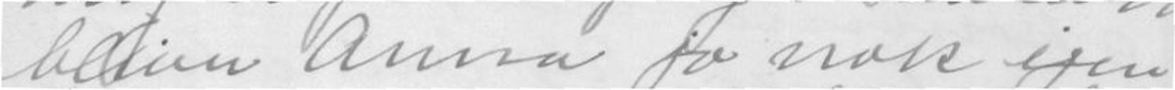bliver Anna jo nok igen
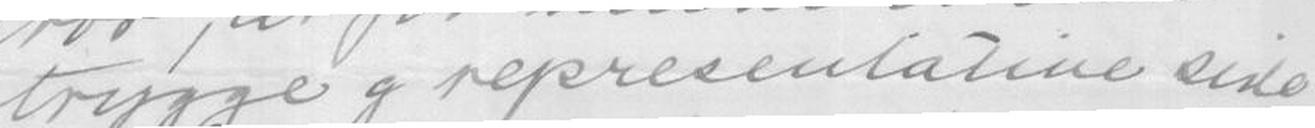trygge og representative side
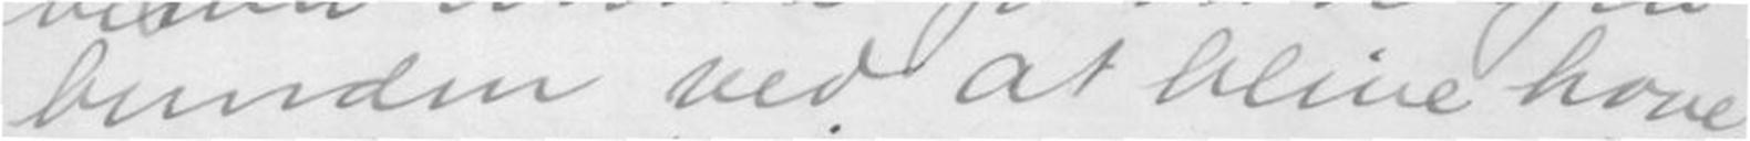bunden ved at blive hove
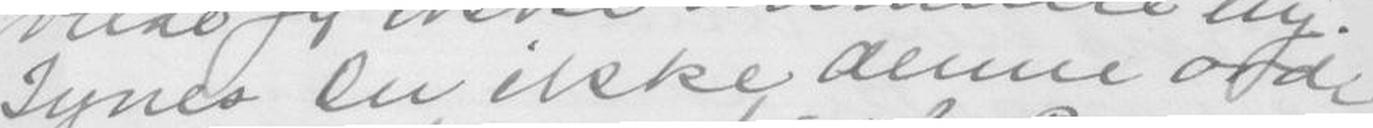Synes Du ikke denne ord-
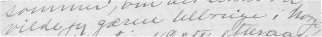vilde jeg gærne tilbringe i Norge,
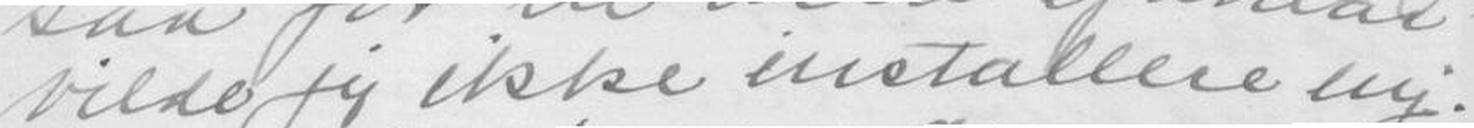vilde jeg ikke installere mig.
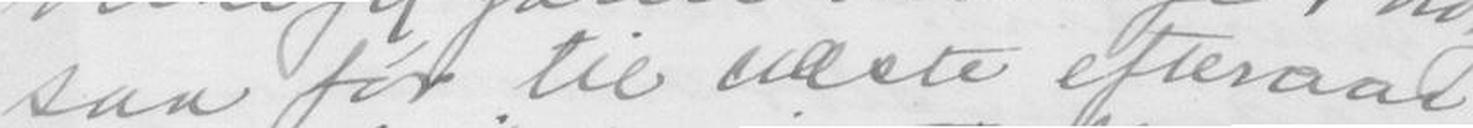saa før til næste efteraar
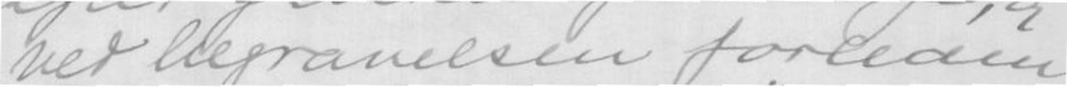ved begravelsen forleden
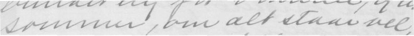sommer, om alt staar vel
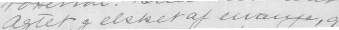Agtet og elsket af mange, og
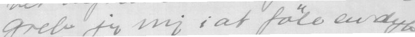greb jeg mig i at føle en dyb
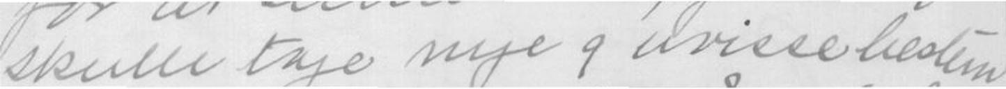skulle tage nye og uvisse bestem-
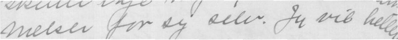melser for sig selv. Jeg vil hellere
